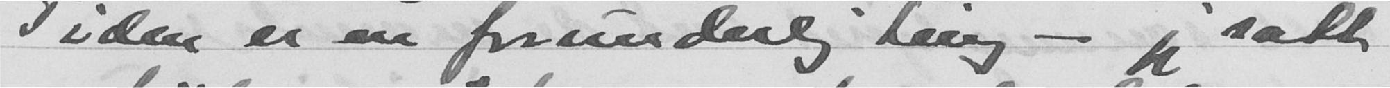Tiden er en forunderlig ting - jeg satt
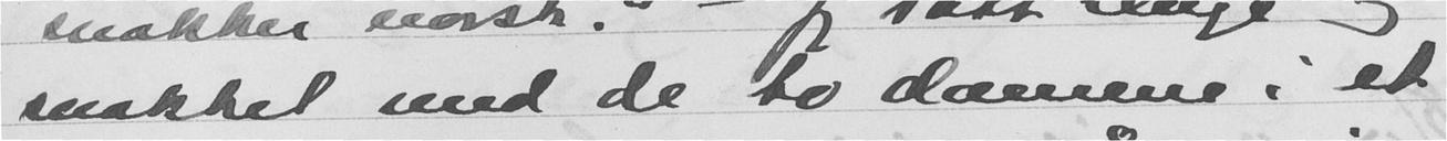snakket med de to damene i et
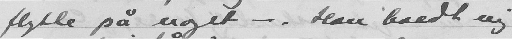flytte på noget -. Han holdt mig
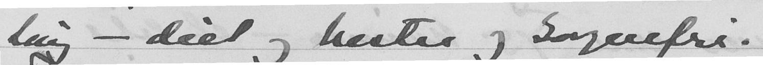ting - drit og hester og Sorgenfri.
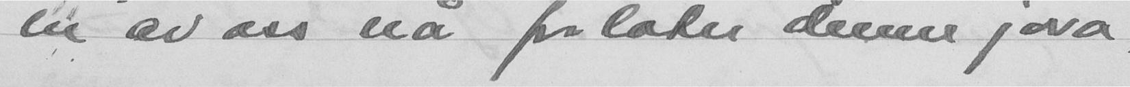en av oss nå forlater denne jora,
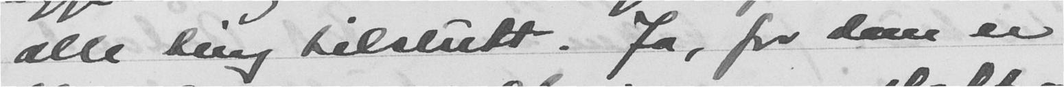alle ting tilslutt. Ja, for dem er
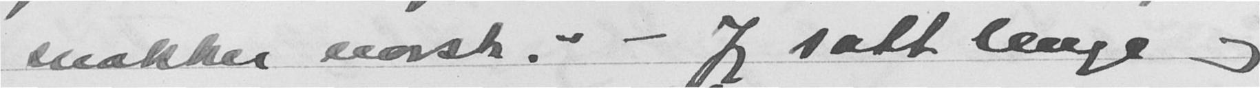snakker norsk." - Jeg satt lenge og
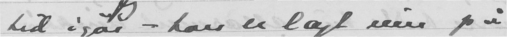tid igår - han er lagt inn på
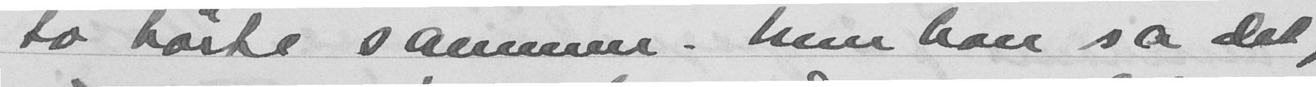to hørte sammen. Men han sa det,
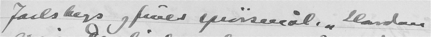Jarlsbergs og fruens spørsmål. "Hvordan
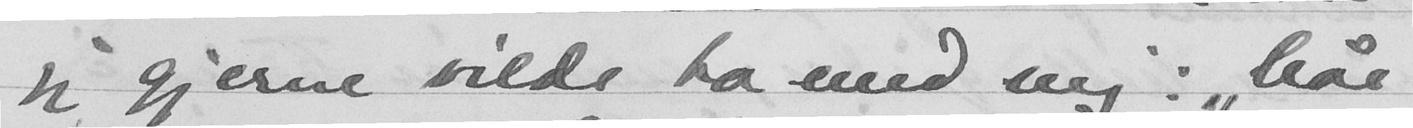jeg gjerne vilde ha med mig: "Når
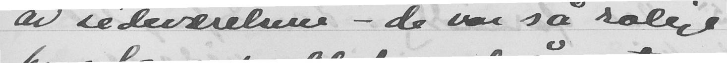av sideværelsene - de var så rolige
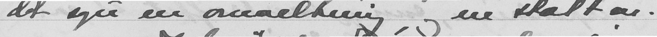det sgu en omveltning og en stolt arv.
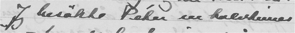Jeg besøkte Peter en halvtimes
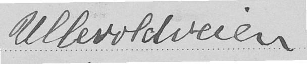Ullevoldveien
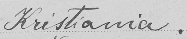Kristiania.
